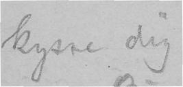kysse dig
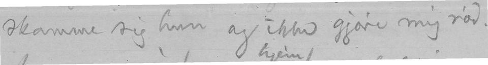skamme sig hun og ikke gjøre mig rød.
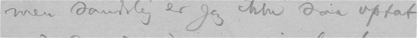men sandelig er jeg ikke saa optat
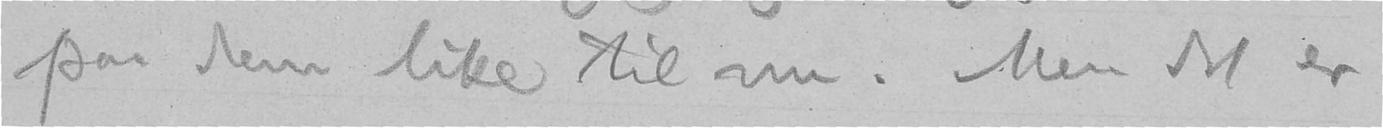paa dem like til nu. Men det er
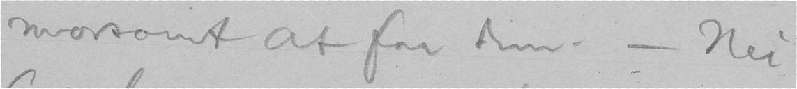morsomt at faa dem. - Nei
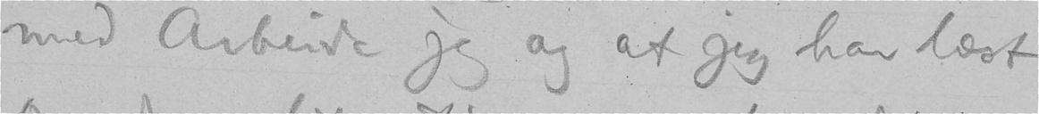med Arbeide jeg og at jeg har læst
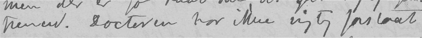fremad. Docteren har ikke rigtig forstaat
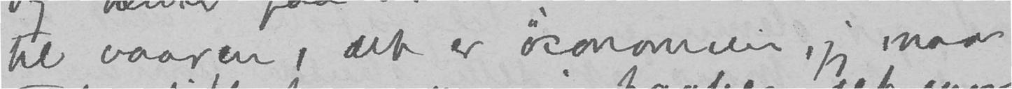til vaaren, det er øconomien, jeg maa
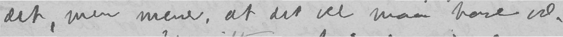det, men mener, at det vel maa have væ-
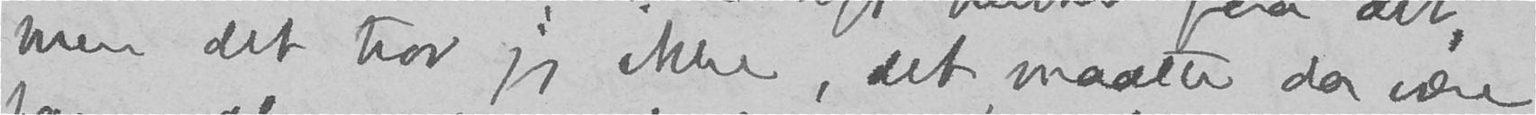men det tror jeg ikke, det maatte da være
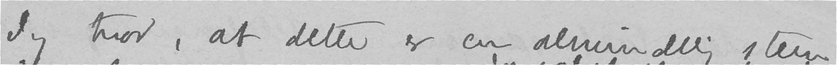Jeg tror, at dette er en almindelig stem-
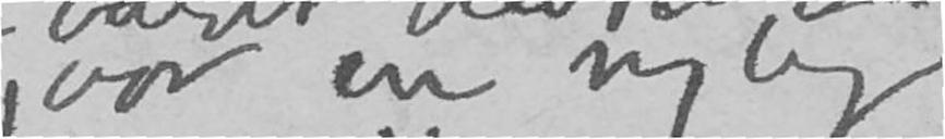var en nylig
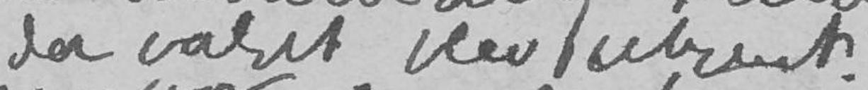da valget blev ulrent
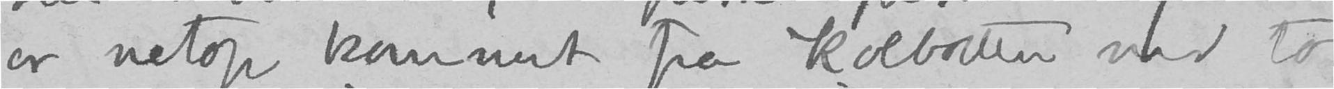er netop kommet fra Kolboten med to
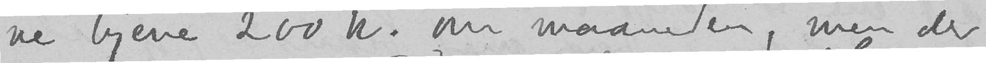ne tjene 200 k. om maanden, men der
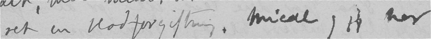ret en blodforgiftning. Smieal og jeg har
